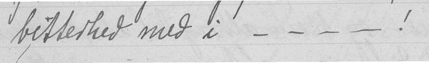bitterhed med i - - - - !
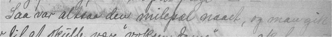Saa var altsaa den milepæl naaet, og man gik
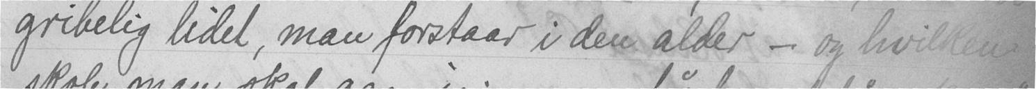gribelig lidet, man forstaar i den alder - og hvilken
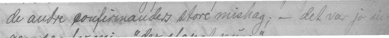de andre confirmanders store mishag; - det var jo in-
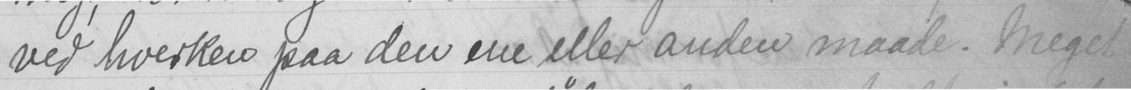ved hverken paa den ene eller anden maade. Meget
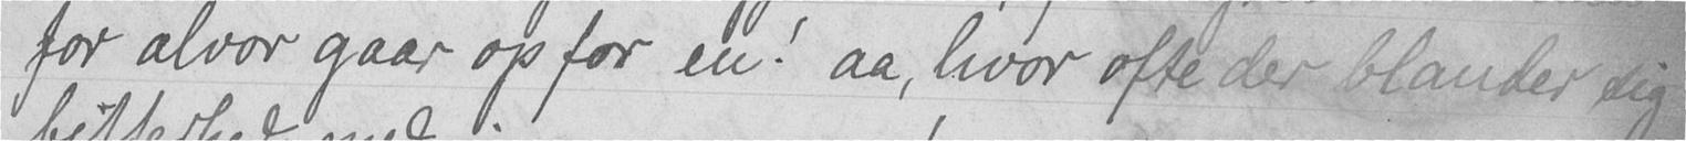for alvor gaar op for en! aa, hvor ofte der blander sig
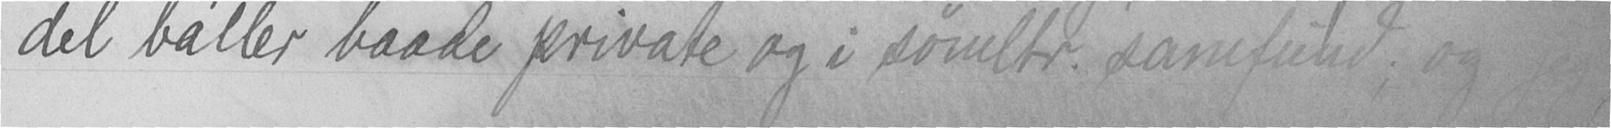del baller baade private og i sømlfr. samfund; og jeg
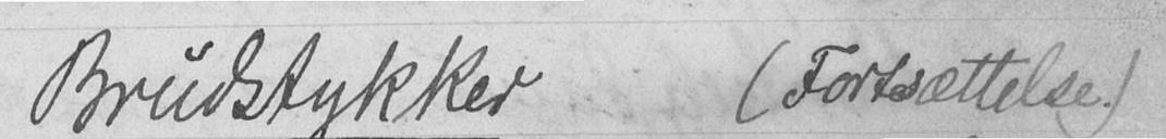Brudstykker - (Fortsættelse.)
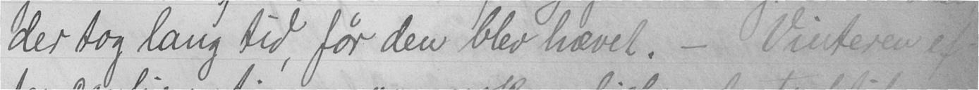der tog lang tid, før den blev hævet. - Vinteren ef-
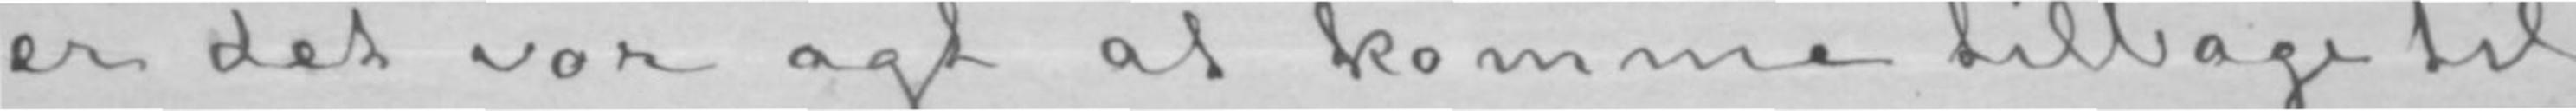er det vor agt at komme tilbage til
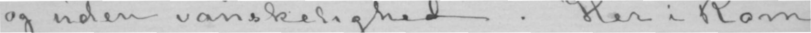og uden vanskelighed. Her i Rom
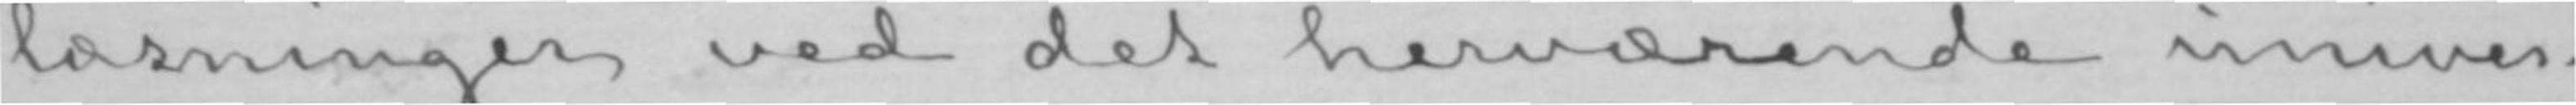læsninger ved det herværende univer-
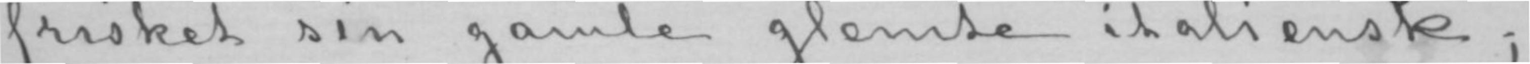frisket sin gamle glemte italiensk;
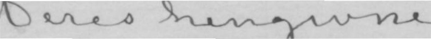Deres hengivne
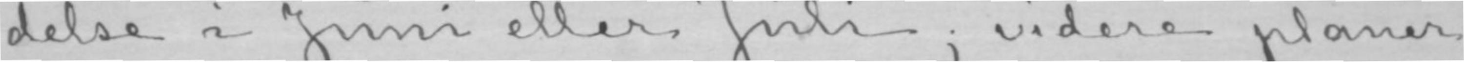delse i Juni eller Juli; videre planer
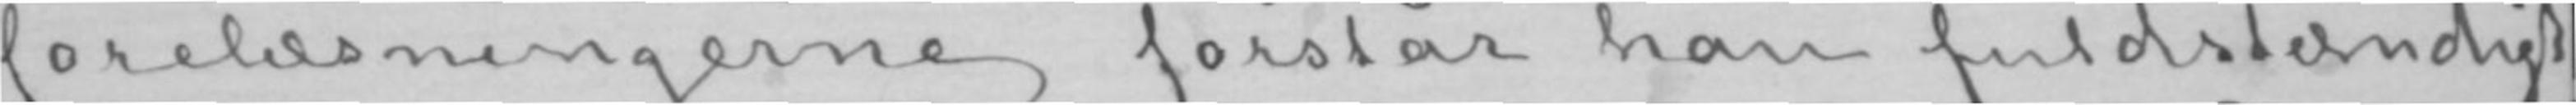forelæsningerne forstår han fuldstændigt
